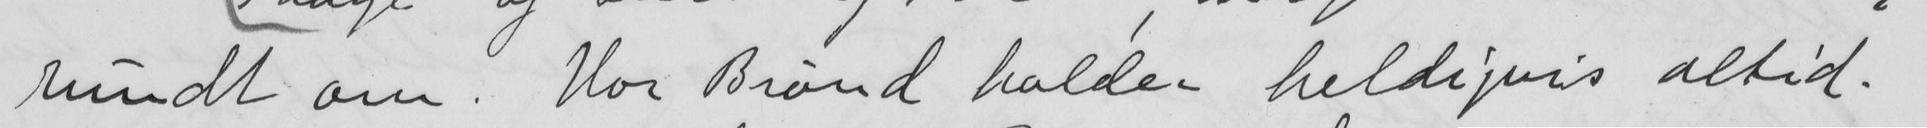rundt om. Vor Brønd holder heldigvis altid.
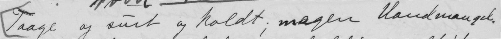Taage og surt og koldt; megen Vandmangel
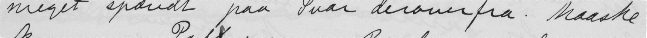meget spændt paa Svar deroverfra. Maaske
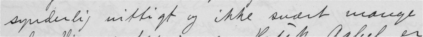synderlig vittigt og ikke svært mange
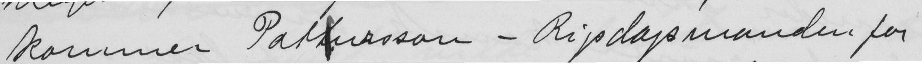kommer Pattursson - Rigsdagsmanden for
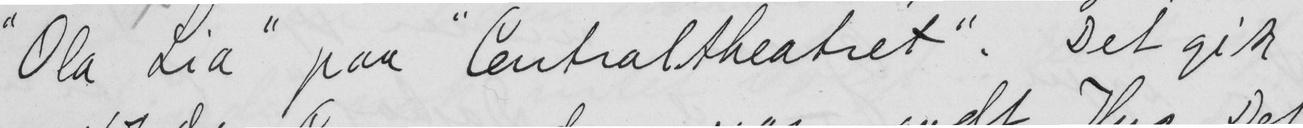"Ola Lia" paa "Centraltheatret". Det gik
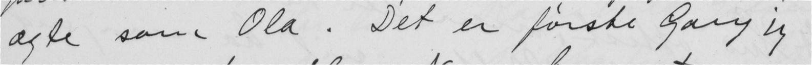ægte som Ola. Det er første Gang Jeg
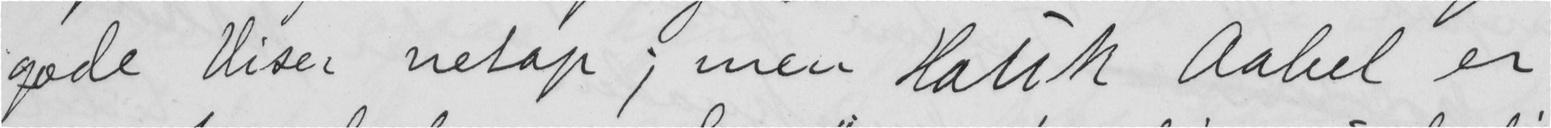gode Viser netop; men Hauk Aabel er
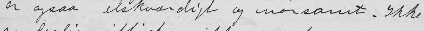er ogsaa elskværdigt og morsomt. Ikke
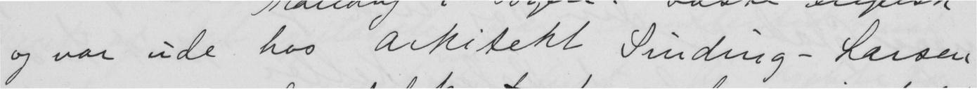og var ude hos Arkitekt Sinding-Larsen
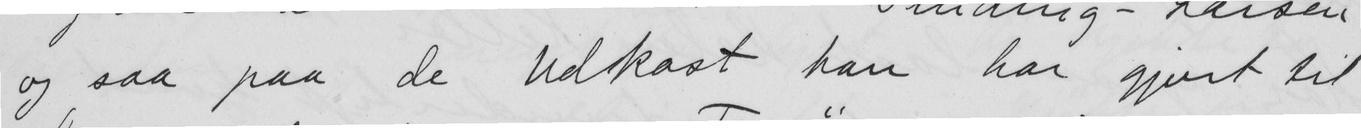og saa paa de Udkast han har gjort til
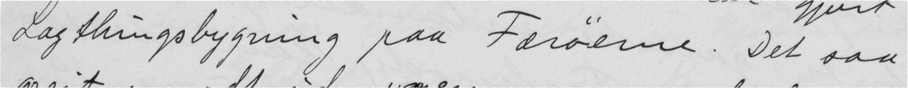Lagthingsbygning paa Færøerne. Det saa
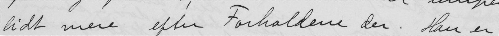lidt mere efter Forholdene der. Han er
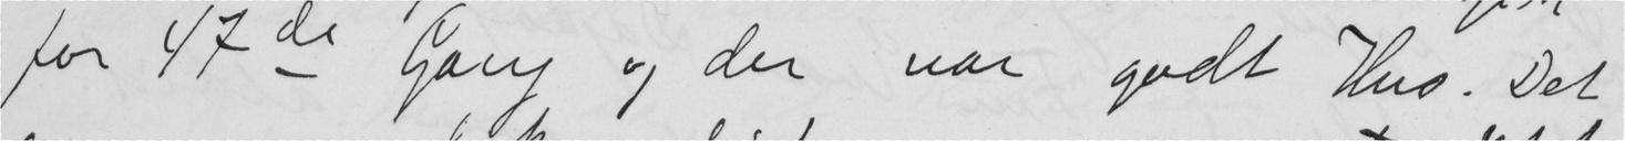for 47de Gans og der var godt Hus. Det
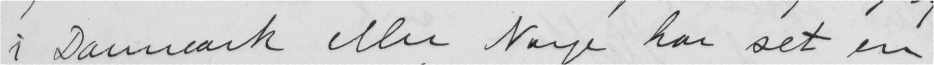i Danmark eller Norge har set en
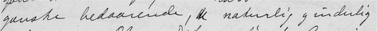ganske bedaarende,  naturlig og inderlig
